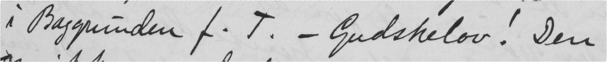i Baggrunden f. T. - Gudskelov! Den
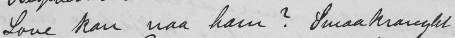Love kan naa ham? Smaakranglet
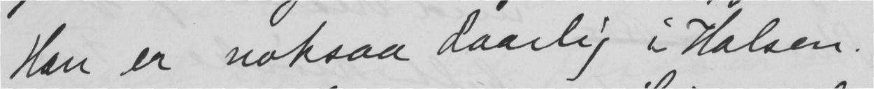Han er noksaa daarlig i Halsen.
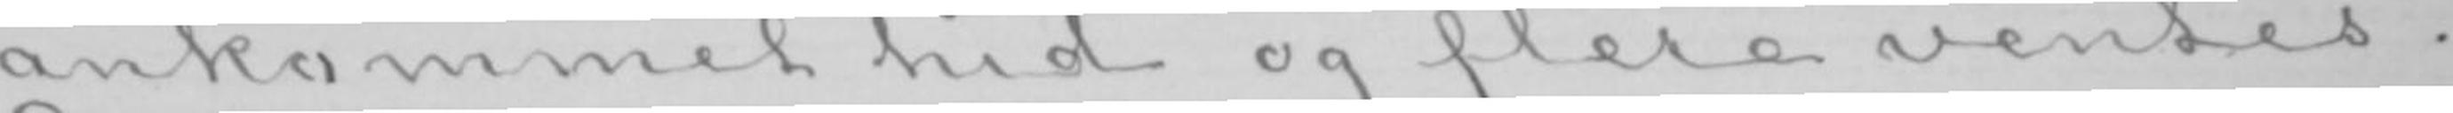ankommet hid og flere ventes.
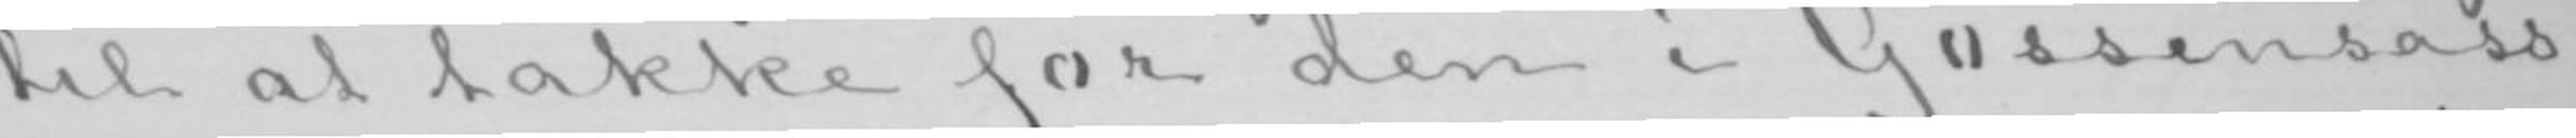til at takke for den i Gossensass
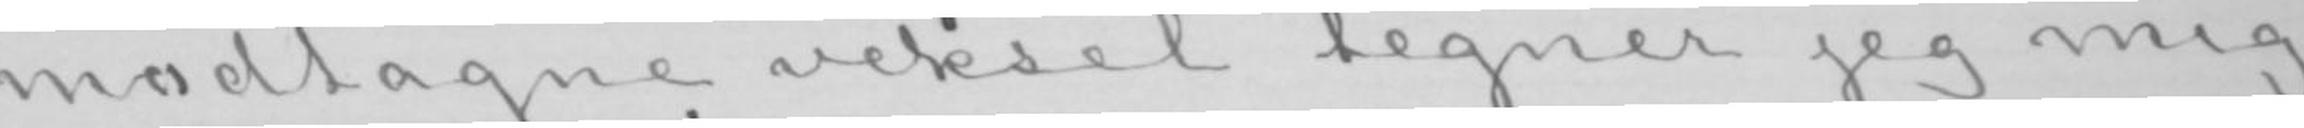modtagne veksel tegner jeg mig
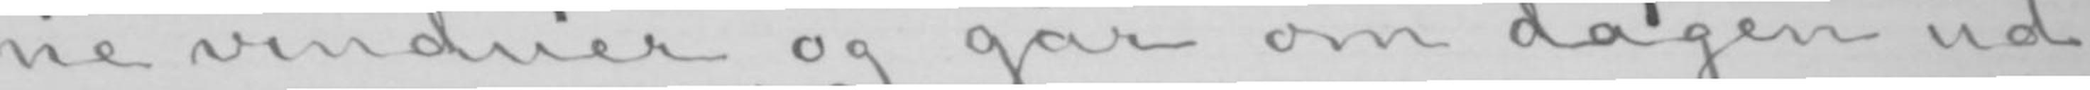ne vinduer og går om dagen ud
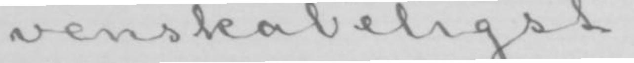venskabeligst
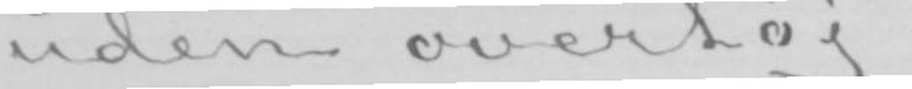uden overtøj.
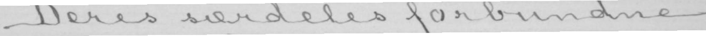Deres særdeles forbundne
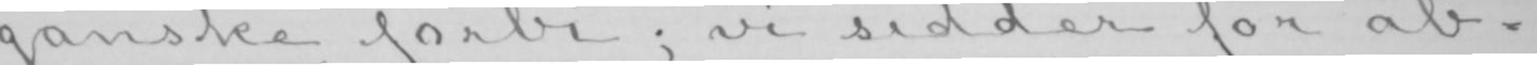ganske forbi; vi sidder for åb-
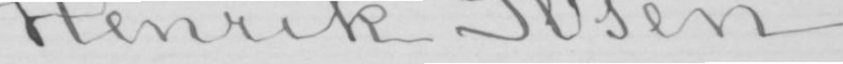Henrik Ibsen.
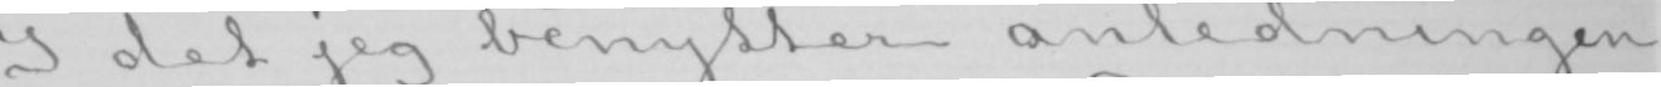I det jeg benytter anledningen
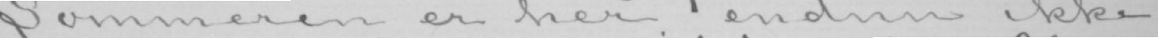Sommeren er her endnu ikke
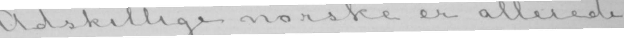Adskillige norske er allerede
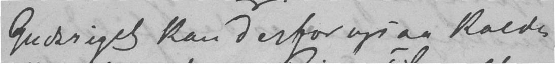Gudsriget kan derfor ogsaa kaldes
